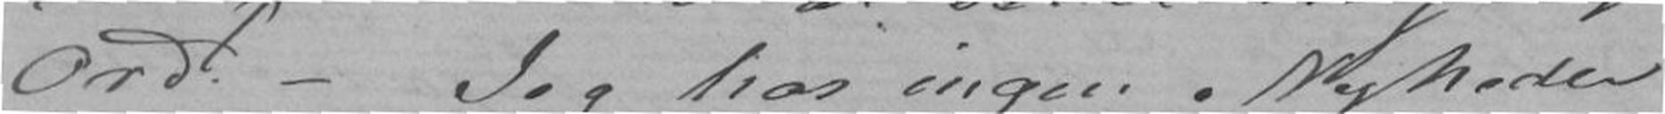Ord. - Jeg har ingen Nyheder
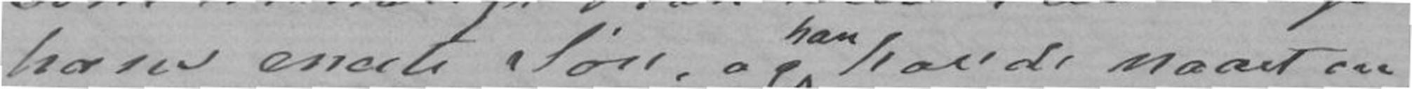hans eneste Søn, og han havde naaet en
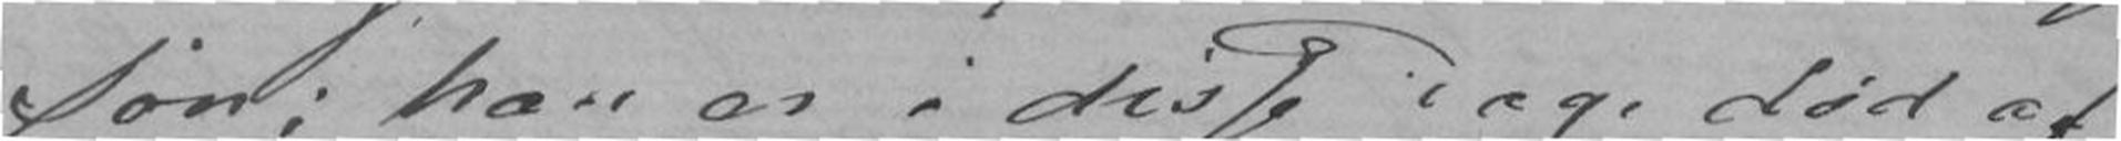Søn; han er i disse Dage død af
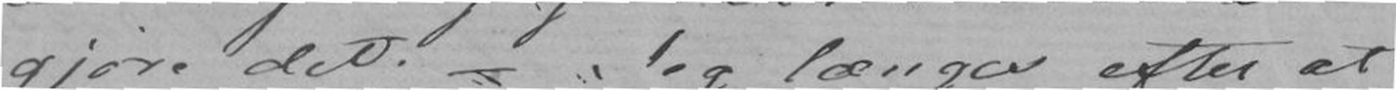gjøre det. Jeg længes efter at
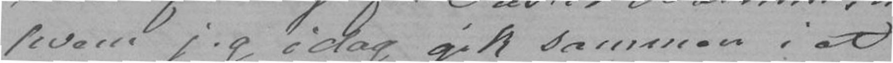hvem jeg idag gik sammen i et
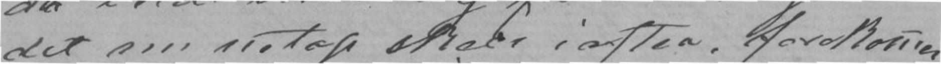det nu netop skede iaften, foreko`mer
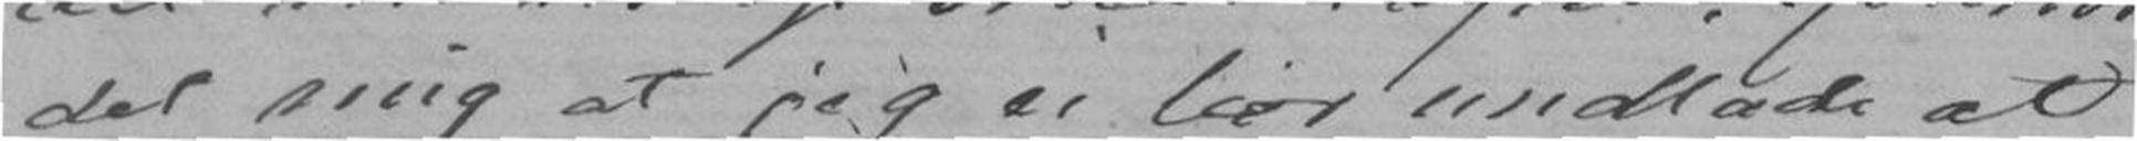det nig at jeg ei bør undlade at
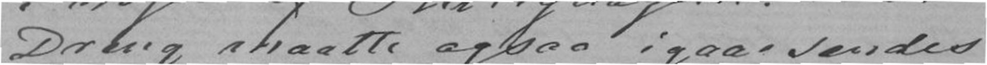Dreng maatte ogsaa igaar sendes
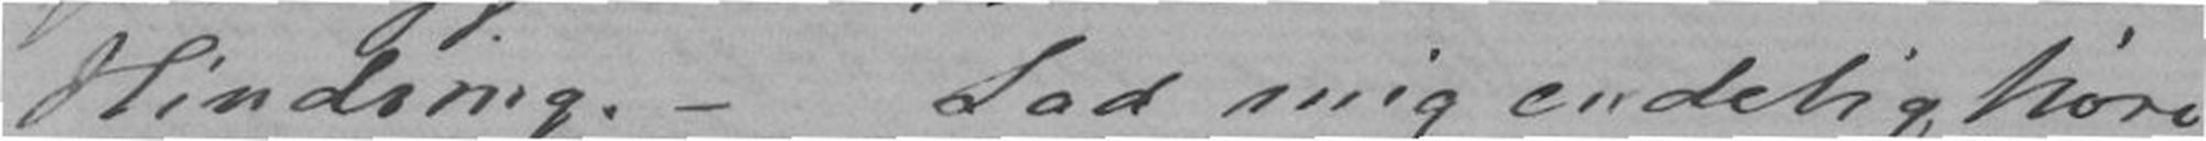Hindring. - Lad mig endelig høre
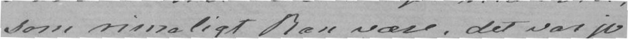som rimeligt kan være, det var jo
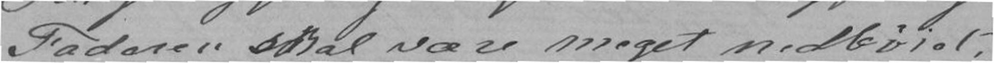Faderen skal være meget nedbøiet,
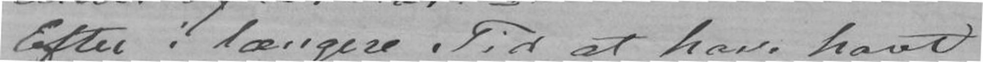Efter i længere Tid at have havt
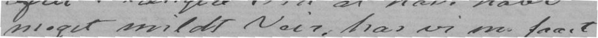meget mildt Veir, har vi nu faaet
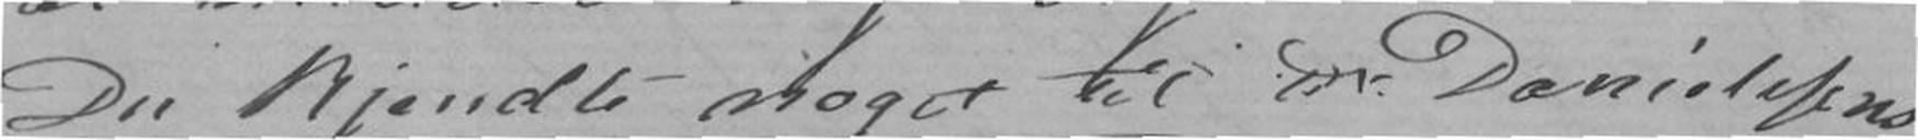Du kjendte noget til Dr. Danielssens
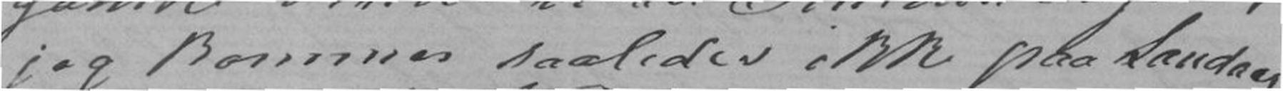jeg kommer saaledes ikke paa Landaas
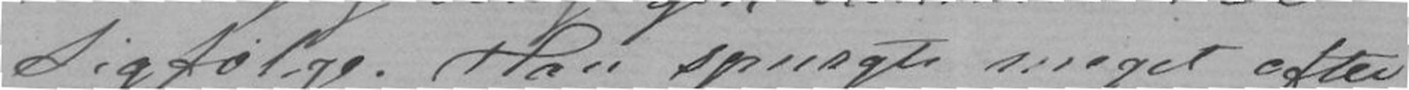Ligfølge. Han spurgte meget efter
